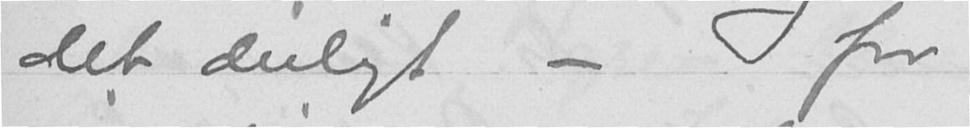det deiligt - for
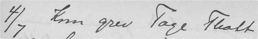4/7 Kom grev Tage Thott
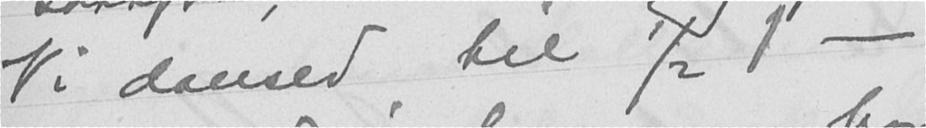Vi dansed til ½ 1 -
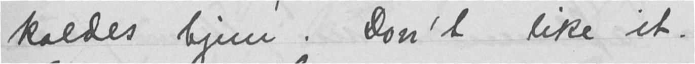kaldes hjem. Don't like it.
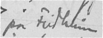paa Fridheim
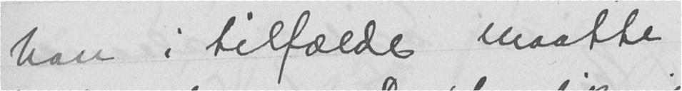han i tilfælde maatte
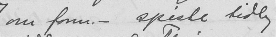om form. - spiste tidlig
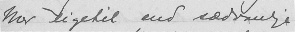Mer ligetil end sædvanlige
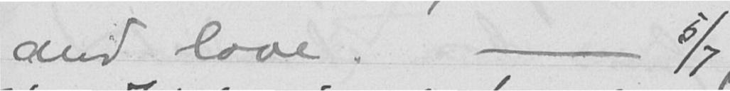and love. - 5/7
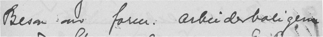Besaa om form. arbeiderboligene
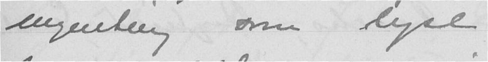ingenting som lyse
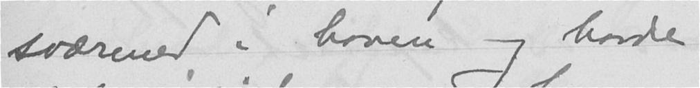sværmed i haven og havde
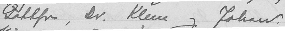Gottfr., Dr. Klem og Johan.
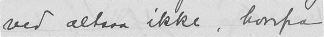ved altsaa ikke, hvorfra
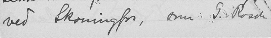ved Skoningfos, som G. Rasch
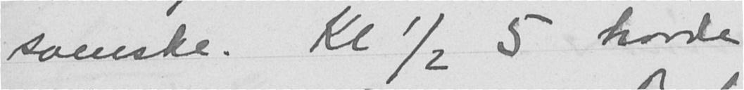svenske. Kl ½ 5 havde
